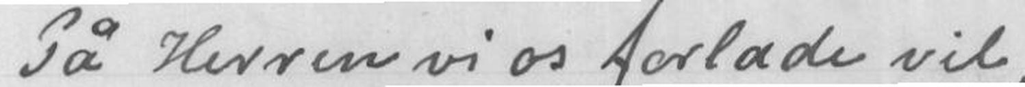På Herren vi os forlade vil,
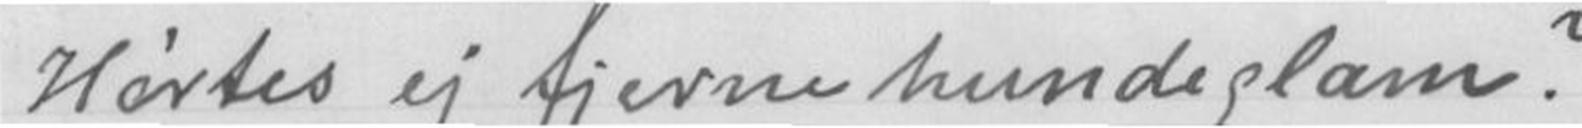Hørtes ej fjerne hundeglam?
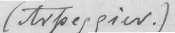(Arpeggier.)
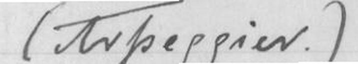(Arpeggier.)
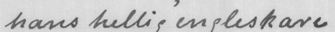hans hellig engleskare
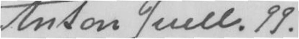Anton Juell. 99.
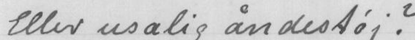Eller usalig åndestøj?
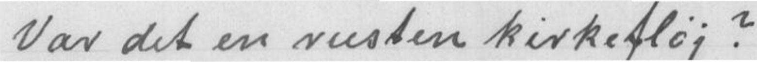Var det en rusten kirkefløj?
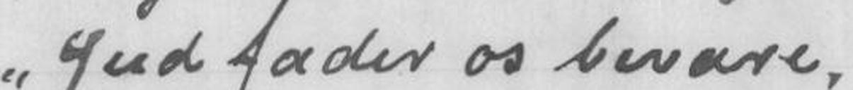Gud fader os bevare,
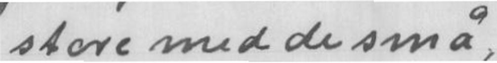store med de små,
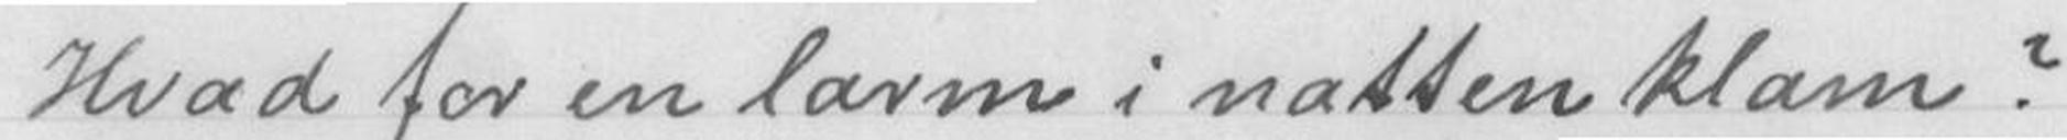Hvad for en larm i natten klam?
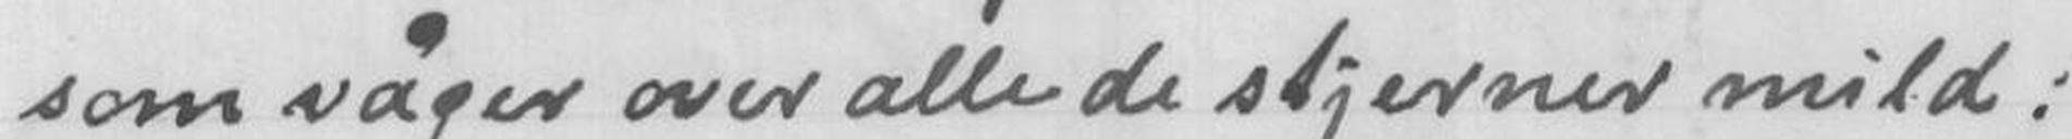som våger over alle de stjerner mild:
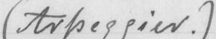(Arpeggier.)
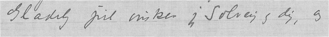Glædelig jul ønsker jeg Solveig og dig, og
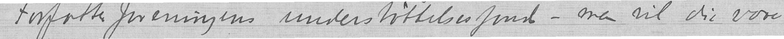Forfatterforeningens understøttelsesfond - men vil du være
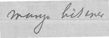mange hilsener
